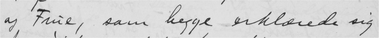og Frue, som begge erklærede sig
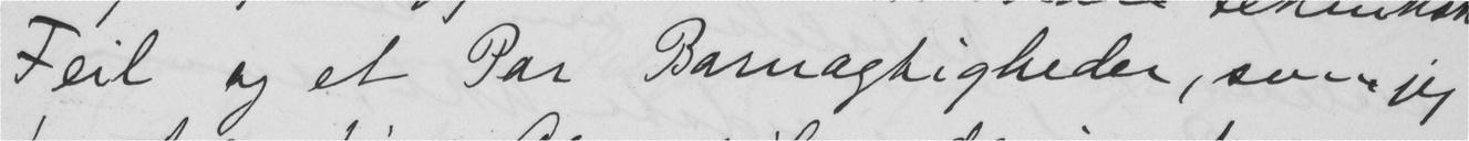Feil og et Par Barnagtigheder, som jeg
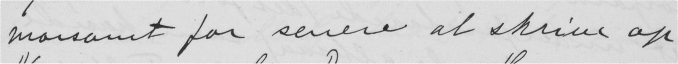morsamt for senere at skrive op
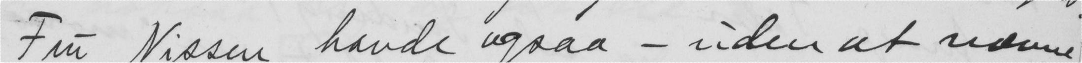Fru Nissen havde ogsaa — uden at nævne
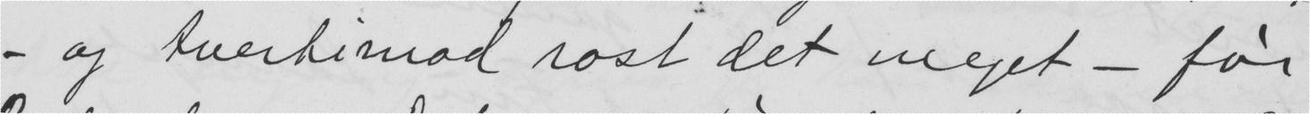- og tvertimod rost det meget — før
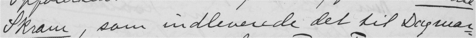Skram, som indleverede det til Dagmar-
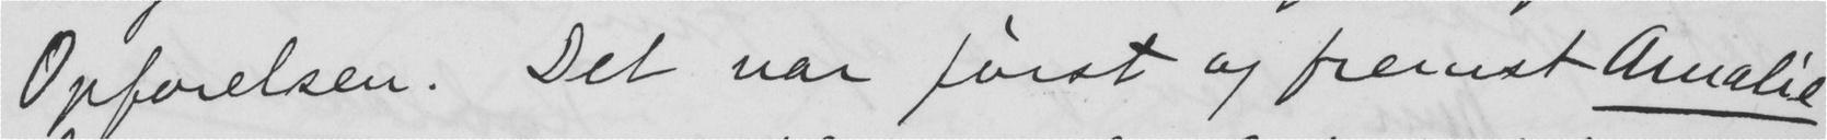Opførelsen. Det var først og fremst Amalie
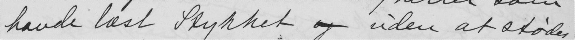havde læst Stykket  uden at stødes
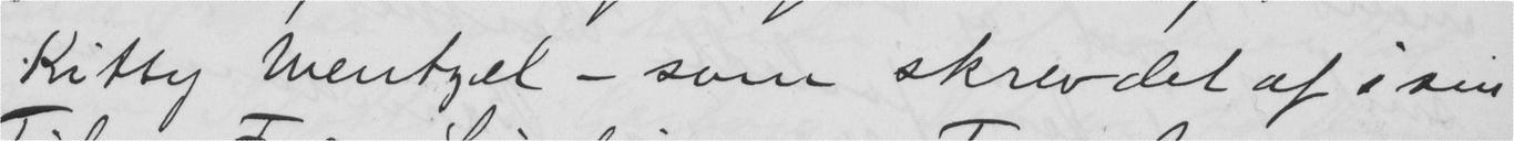Kitty Wentzel - som skrev det af i sin
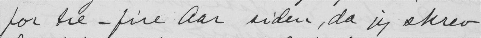for tre - fire Aar siden, da jeg skrev
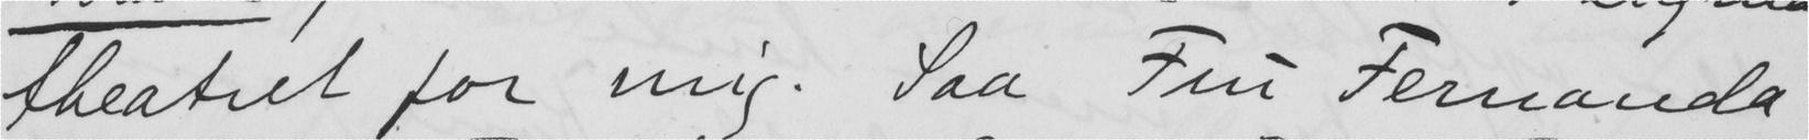Theatret for mig. Saa Fru Fernanda
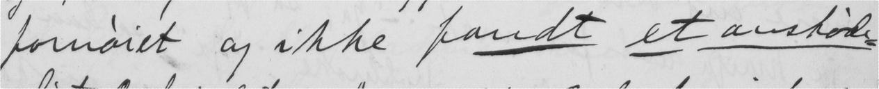fornøiet og ikke fandt et anstøde-
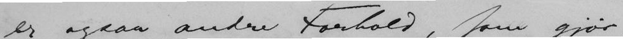er ogsaa andre Forhold, som gjør,
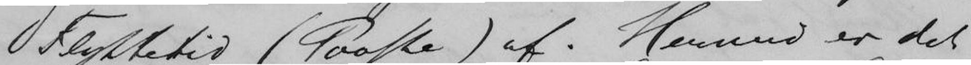Flyttetid (Paaske) af. Hermed er det
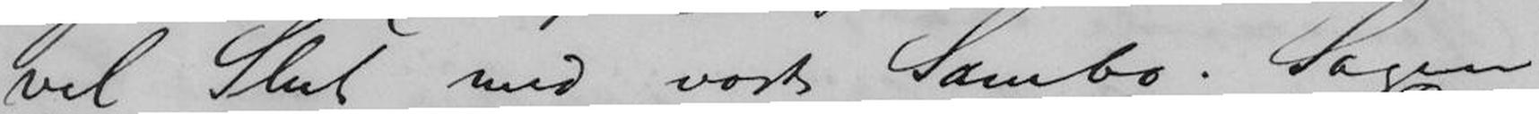vel Slut med vort Sambo. Sagen
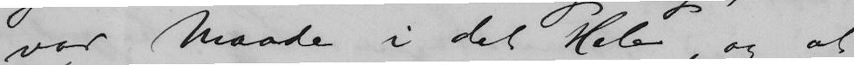vor Maade i det Hele, og at
